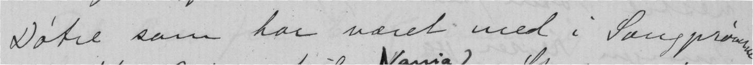Døtre som har været med i Sangprøvene
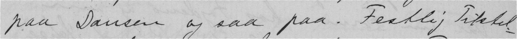paa Dansen og saa paa. Festlig Tilstel-
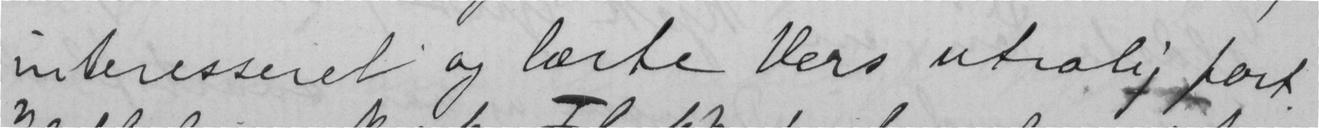interesseret og lærte Vers utrolig fort.
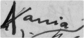Xania
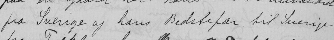fra Sverige og hans Bedstefar til Sverige
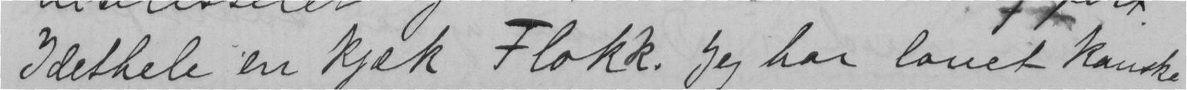Idethele en kjæk Flokk. Jeg har lovet kanske
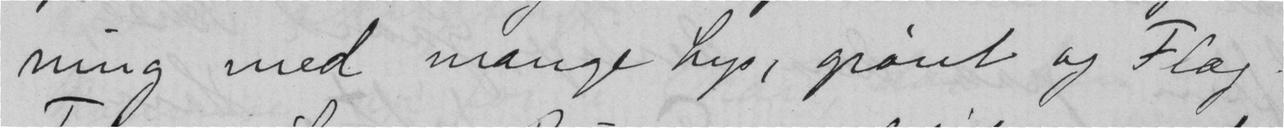ning med mange Lys, grønt og Flag.
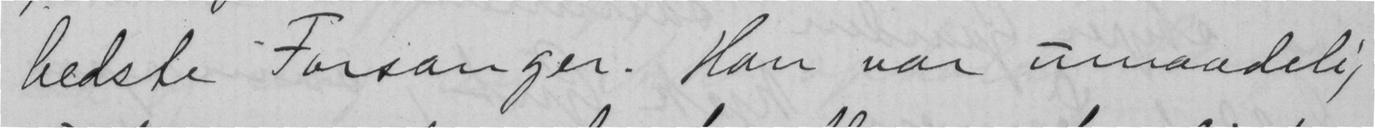bedste Forsanger. Han var umaadelig
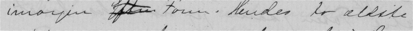imorgen  Form. Hendes to ældste
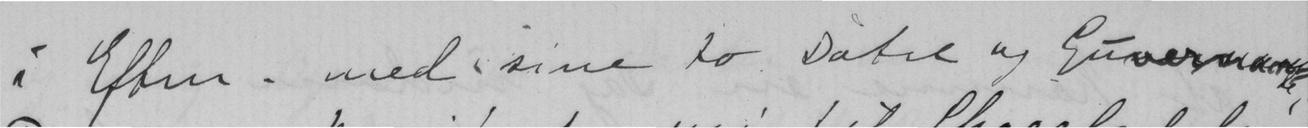i Eften. med i sine to Døtre og Guvernante,
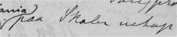paa Skoler netop
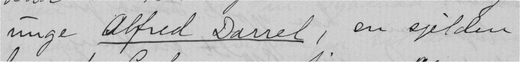unge Alfred Darrel, en sjelden
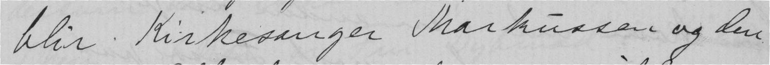blir Kirkesanger Markussen og den
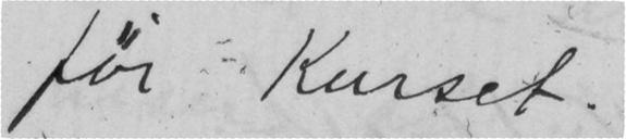før Kurset.
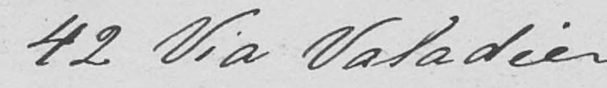42 Via Valadier
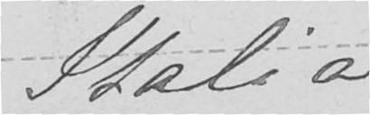Italia
